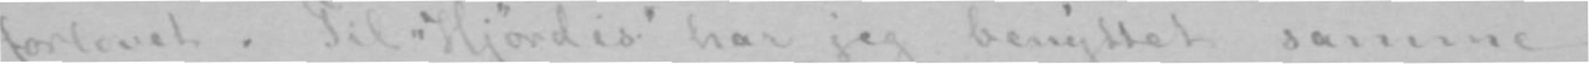forlovet. Til «Hjørdis» har jeg benyttet samme
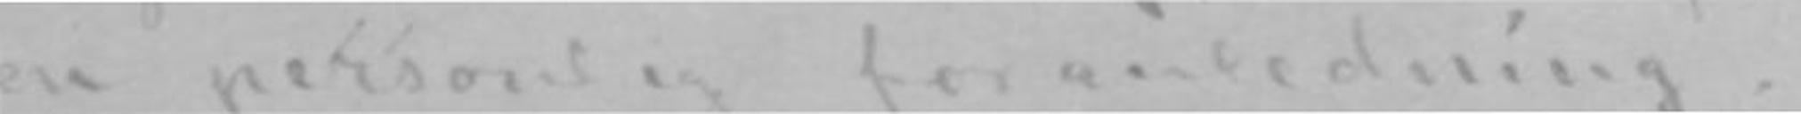en personlig foranledning.
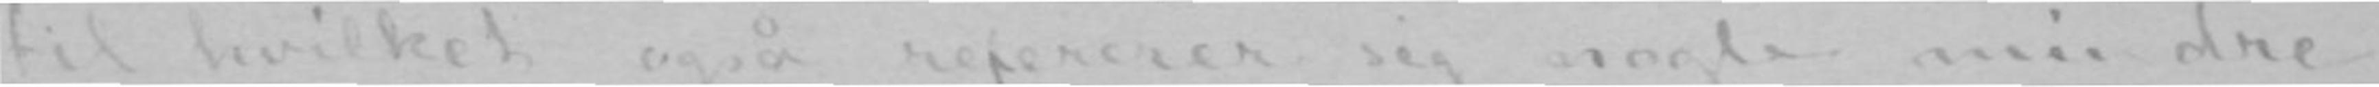til hvilket også refererer sig nogle mindre
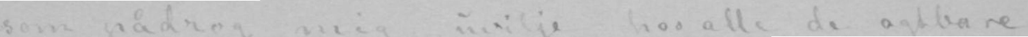som pådrog mig uvilje hos alle de agtbare
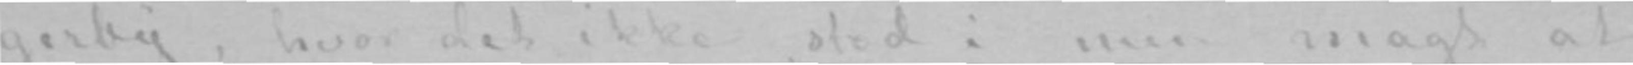gerby, hvor det ikke stod i min magt at
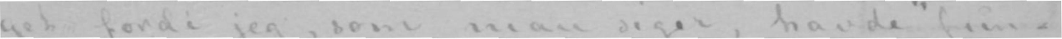get fordi jeg, som man siger, havde «fun-
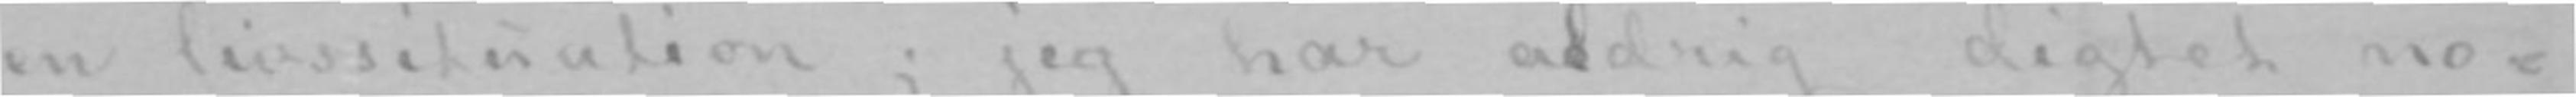en livssituation; jeg har aldrigad digtet no-
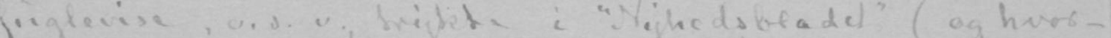fuglevise, o. s. v., trykte i «Nyhedsbladet» (og hvor-
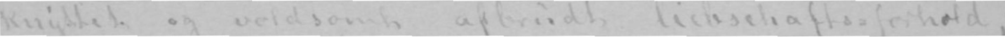knyttet og voldsomt afbrudt liebschafts-forhold,
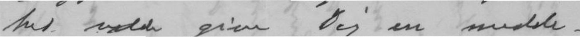bet vilde give Dig en medde-
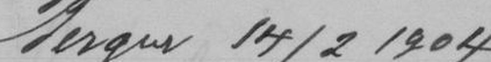Bergen 14/2 1904.
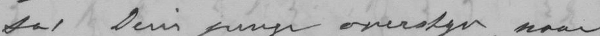sat Dine penger overstyr, naar
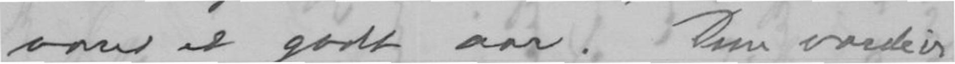været et godt aar. Dine verdier
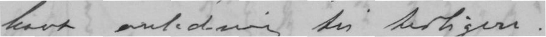havt anledning til tidligere.
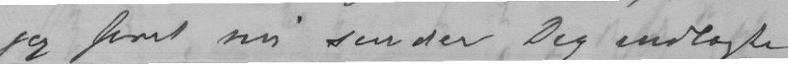jeg først nu sender Dig indlagte
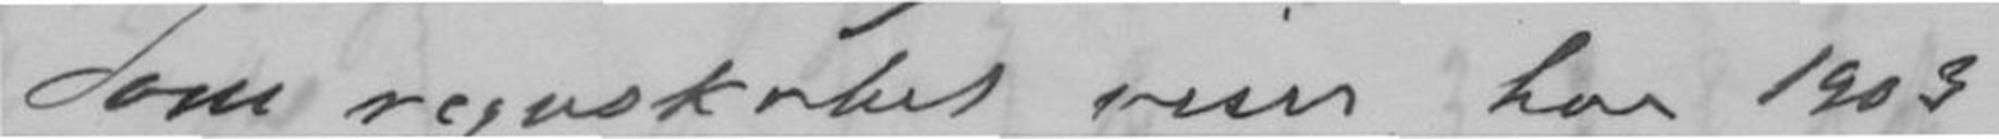Som regnskabet viser, har 1903
